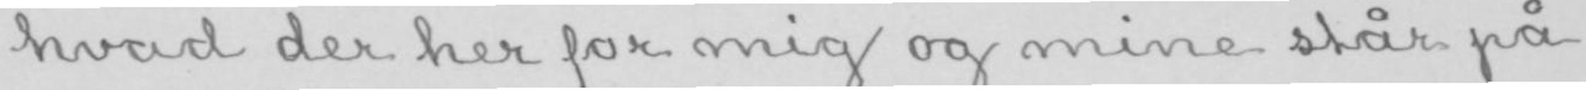hvad der her for mig og mine står på
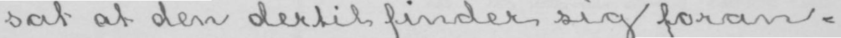sat at den dertil finder sig foran-
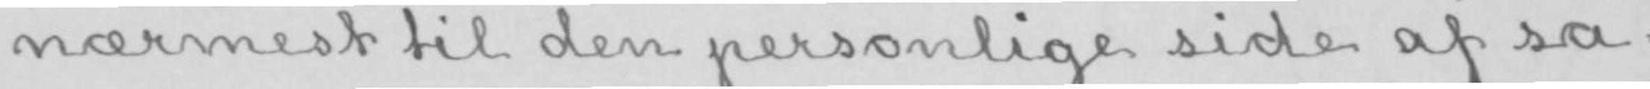nærmest til den personlige side af sa-
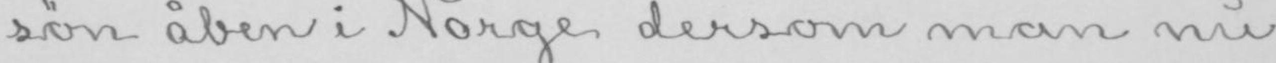søn åben i Norge dersom man nu
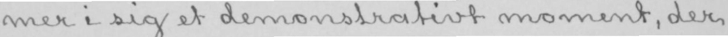mer i sig et demonstrativt moment, der
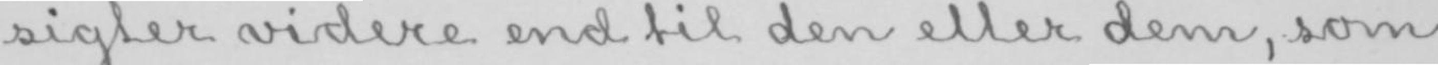sigter videre end til den eller dem, som
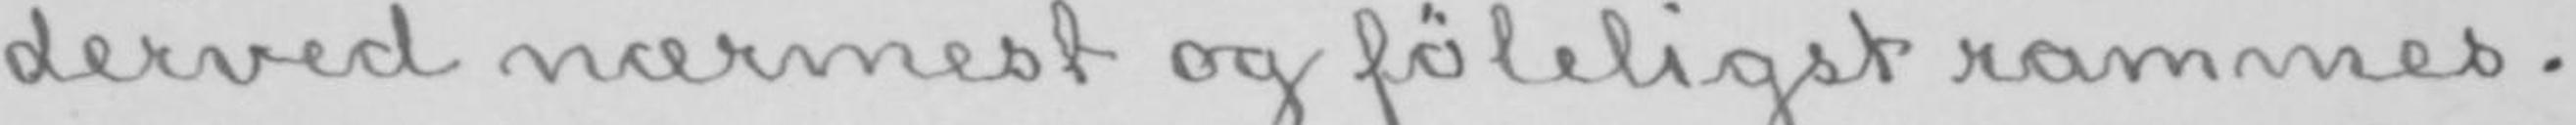derved nærmest og føleligst rammes.
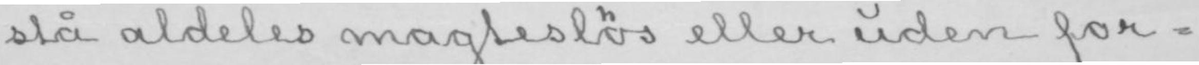stå aldeles magtesløs eller uden for-
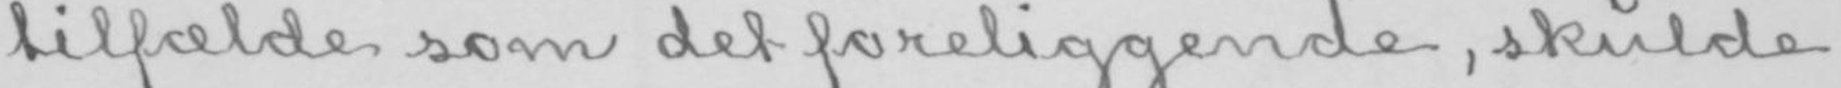tilfælde som det foreliggende, skulde
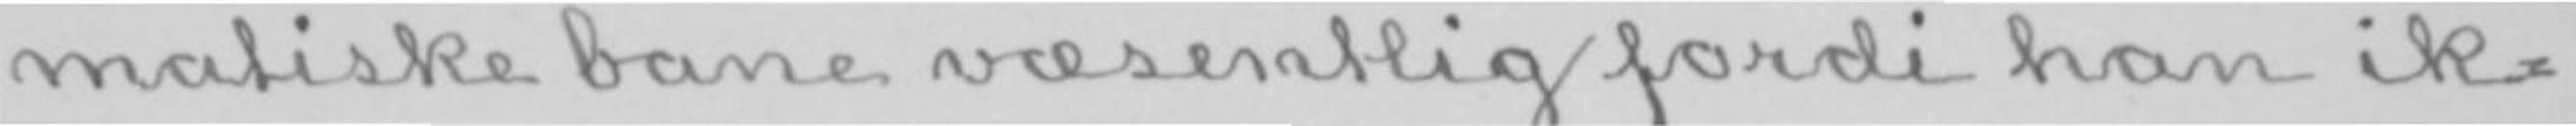matiske bane væsentlig fordi han ik-
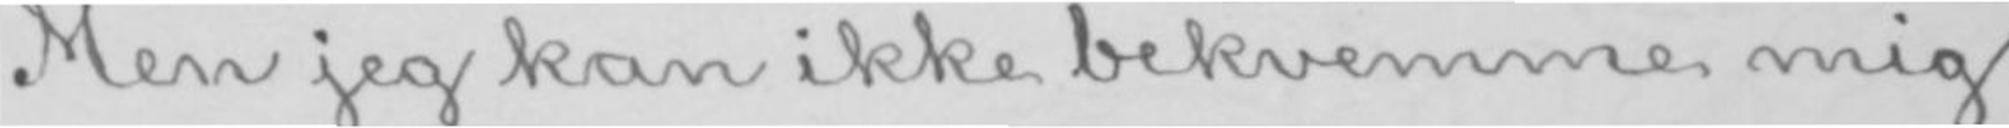Men jeg kan ikke bekvemme mig
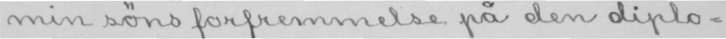min søns forfremmelse på den diplo-
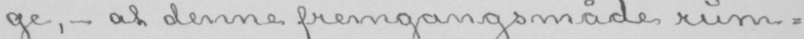ge,- at denne fremgangsmåde rum-
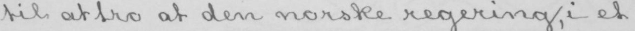til at tro at den norske regering, i et
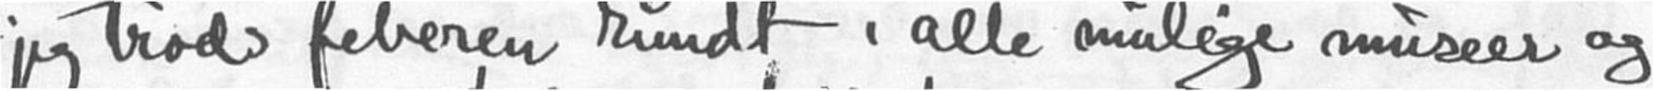jeg trods feberen rundt i alle mulige museer og
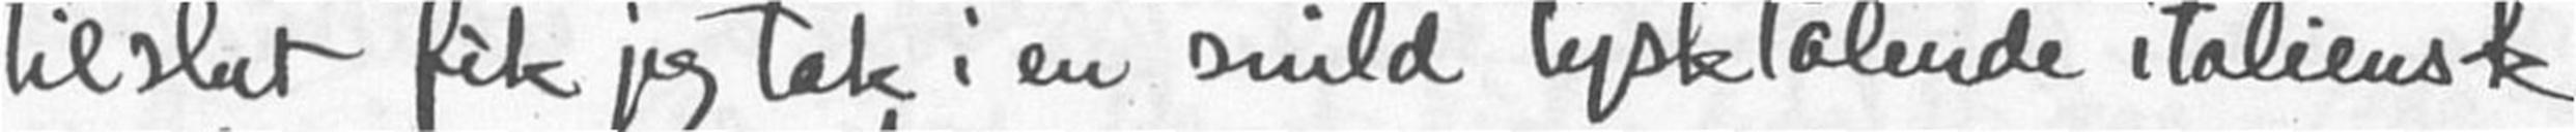tilslut fik jeg tak i en snild tysktalende italiensk
